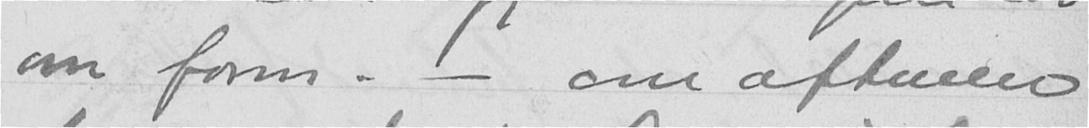om form. - om aftenen
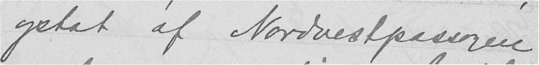optat af Nordvestpassagen
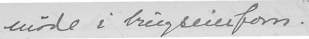møde i brugseierforn.
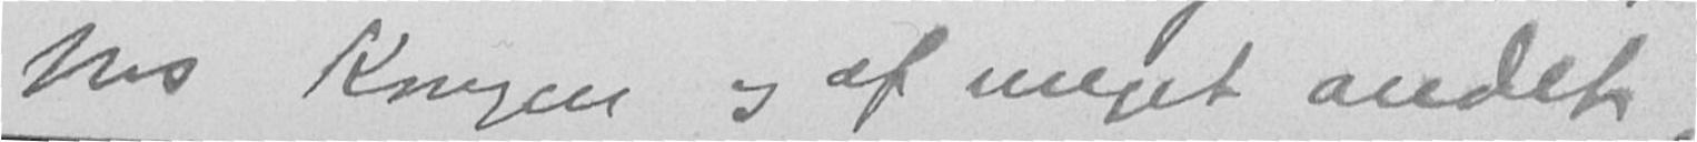hos Kongen og af meget andet.
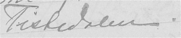Tistedalen.
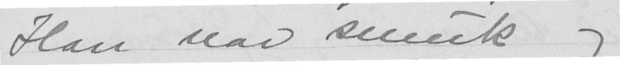Han var smuk og
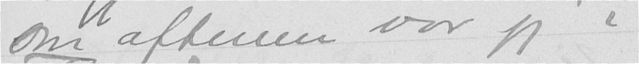Om aftenen var jeg i
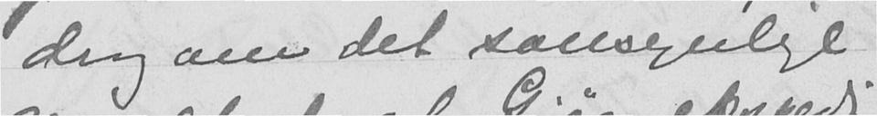drag om det sansynlige
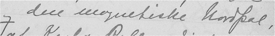og den magnetiske Nordpol,
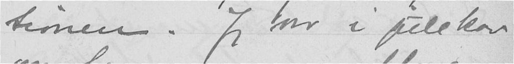tionen. Jeg var i julekor
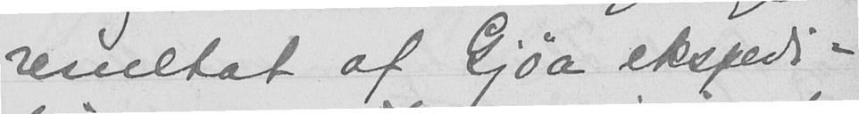resultat af Gjøa ekspedi-
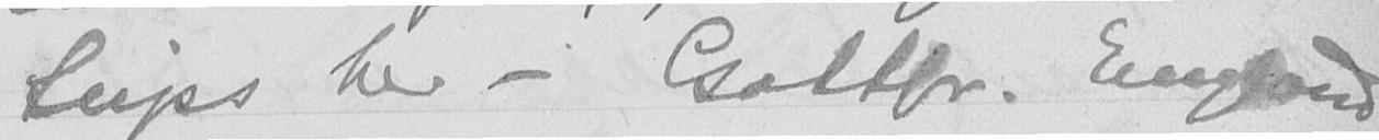Seips her - Gottfr. England
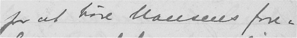for at høre Nansens fore-
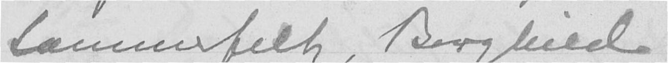Sommerfelts, Borghild
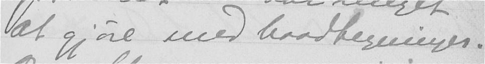at gjøre med baadtegninger.
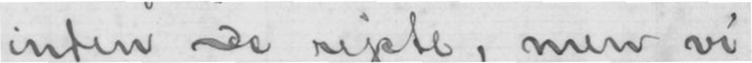inden de rejste, men vi
